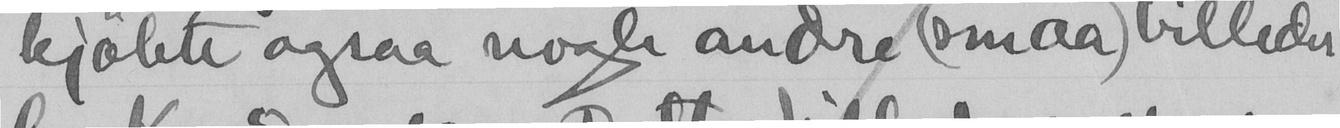kjøbte ogsaa nogle andre (smaa) billeder
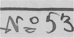No 53
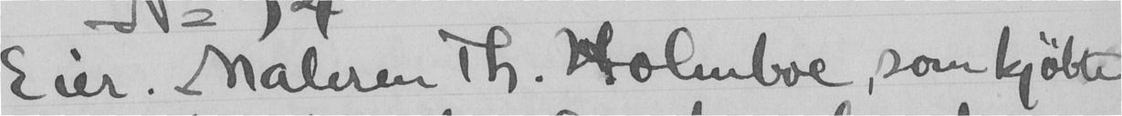Eier: Maleren Th. Holmboe, som kjøbte
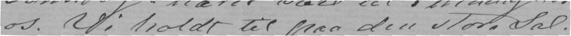os. Vi holdt til paa den store Sal
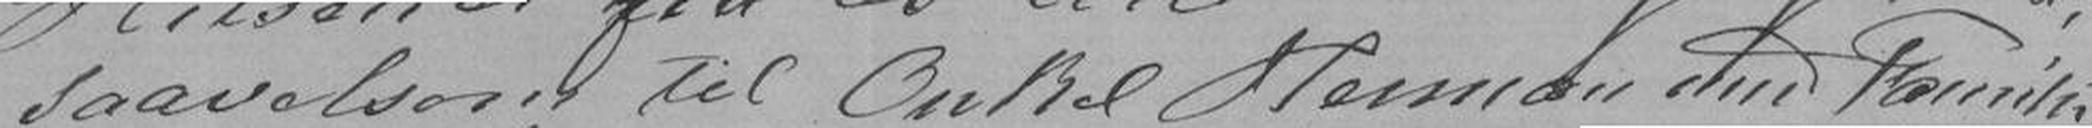saavelsom til Onkel Hermen med Familie,
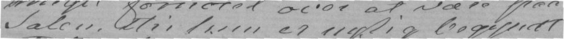Salen, thi hun er nylig begyndt
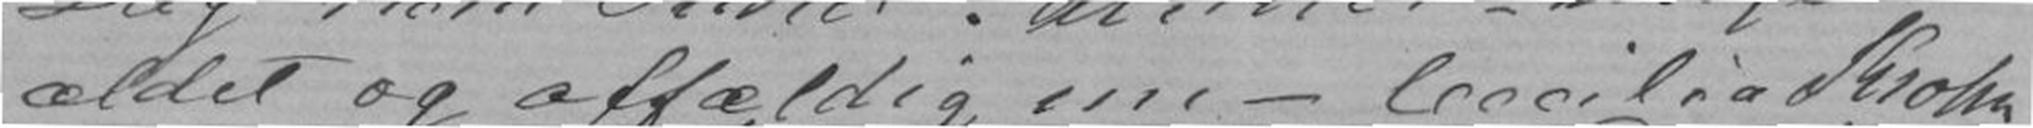ældet og affældig nu - Cecilia Krohn
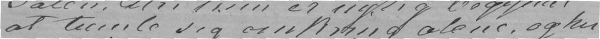at tumle sig omkring alene, og her
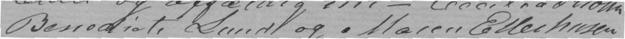Benedicte Lund og Maren Ellerhusen
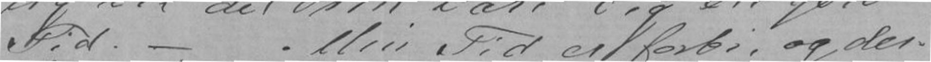Tid. - Min Tid er forbi, og der-
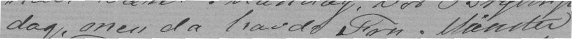dag, men da havde Fru Münster
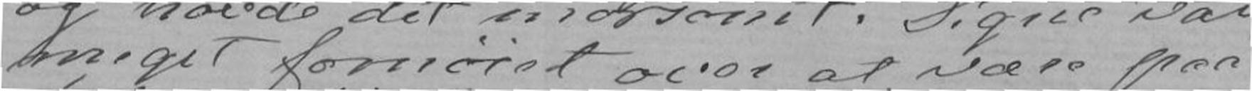meget fornøiet over at være paa
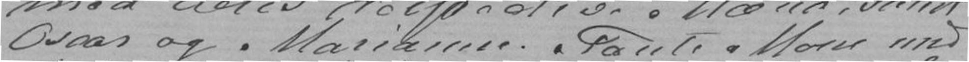Oscar og Marianne. Tante Mone med
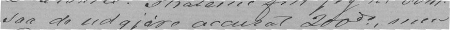saa de udgjør accurat 200de, men
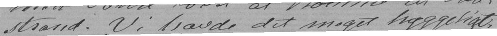strand. Vi havde det meget hyggeligt,
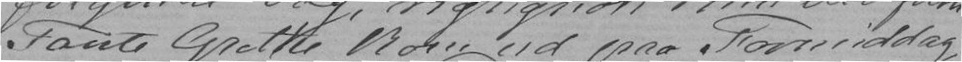Tante Grethe kom ud paa Formiddag
